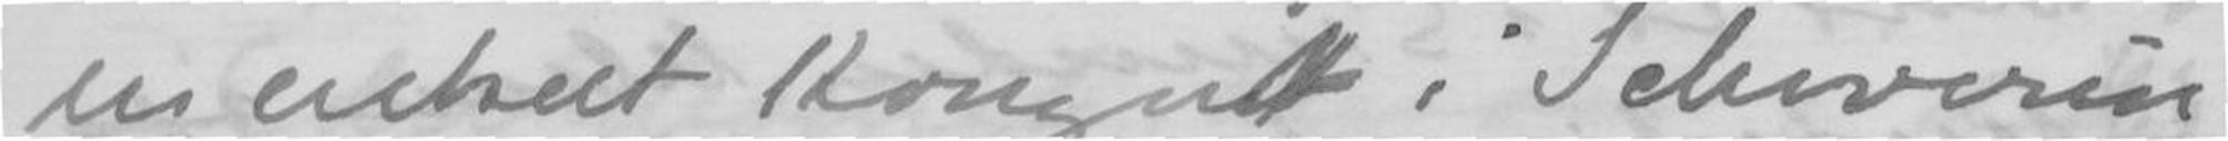en enkelt Konzert i Schwerin
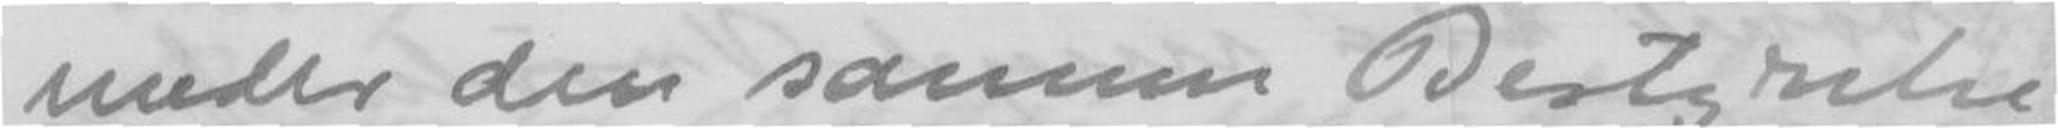under den samme Bestyrelse
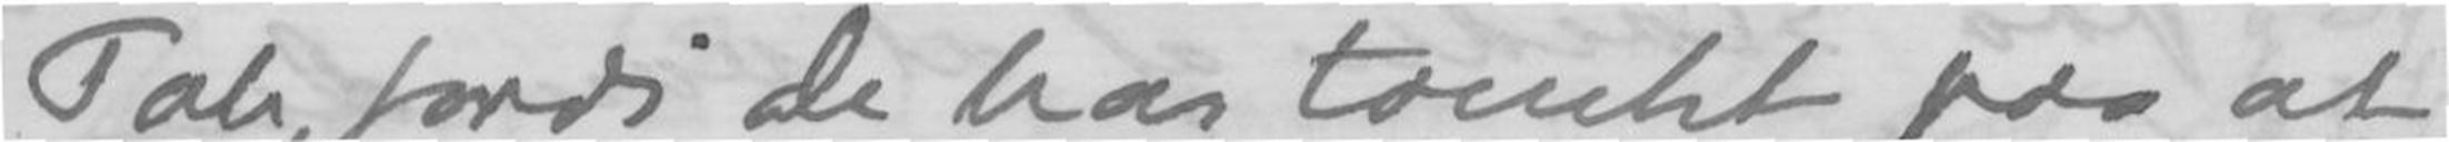Tale, fordi De har taenkt paa at
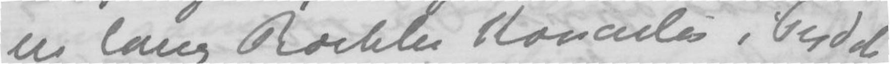en lang Raekke Koncerter i Tydsk-
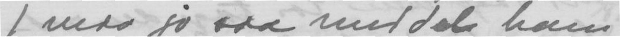J maa jo saa meddele ham
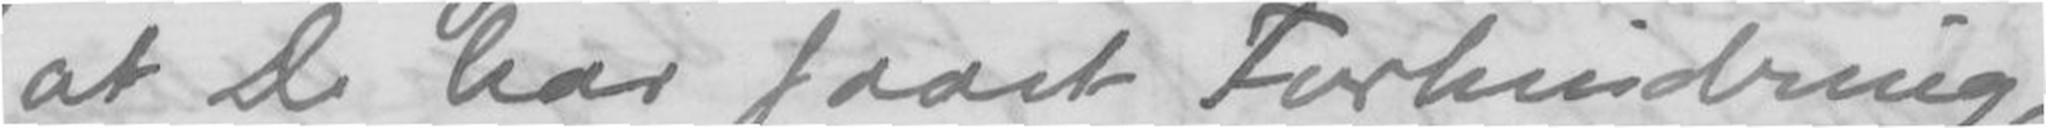at De har faaet Forhindring,
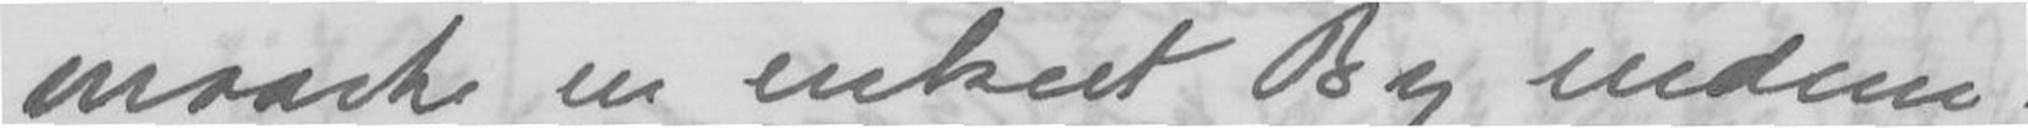maaske en enkelt By endnu.
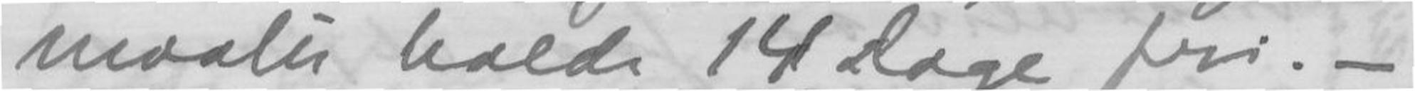maatte halde 14 Dage fri. -
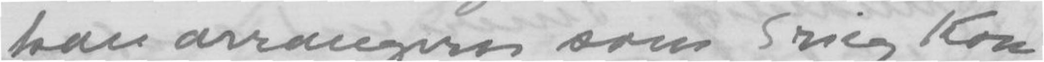kan arrangeres som Grieg Kon-
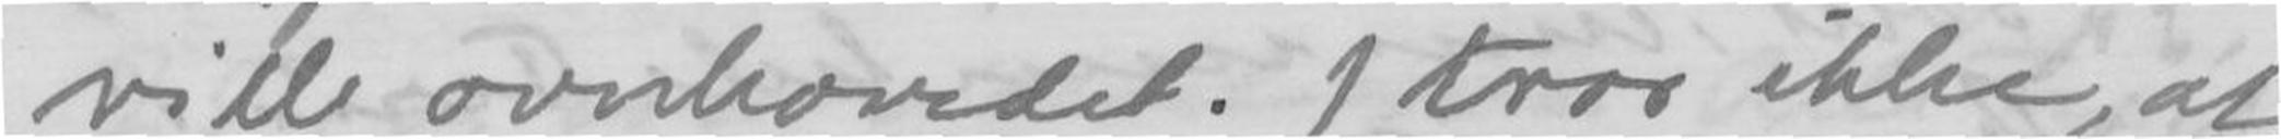ville overhovedet. J tror ikke, at
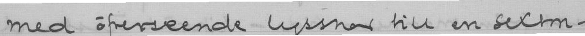med öfverseende lyssner till en sexton-
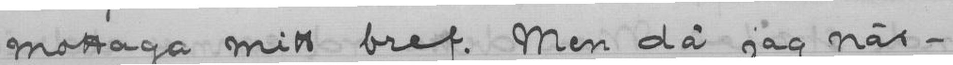mottaga mitt bref. Men då jag när-
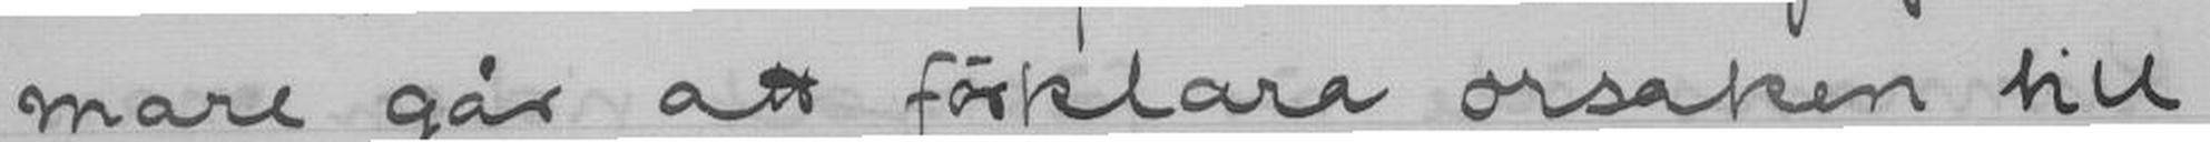mare går att förklara orsaken till
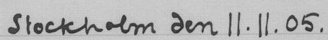Stockholm den 11.11.05.
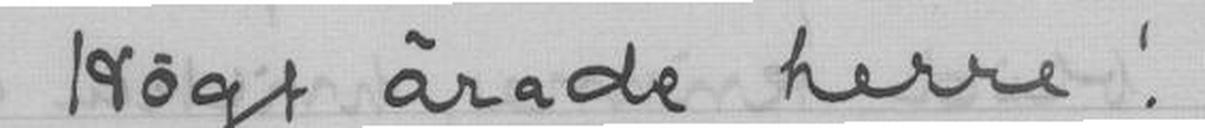Högt ärade herre!
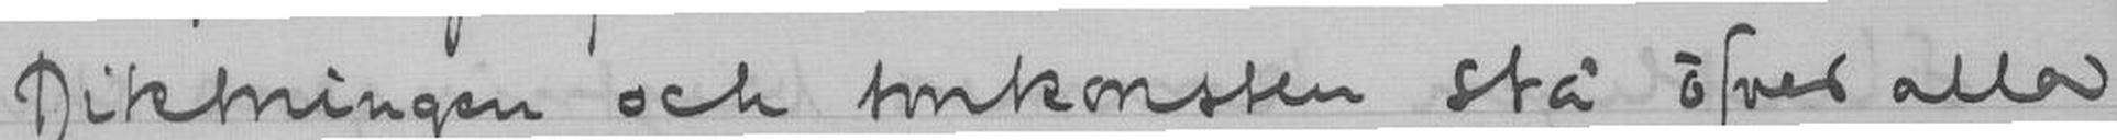Diktningen och tonkonsten stå öfver alla
